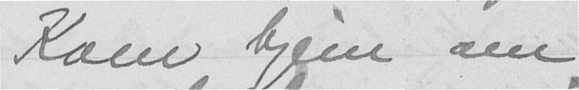Kom hjem om
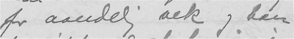for aandelig vek og han
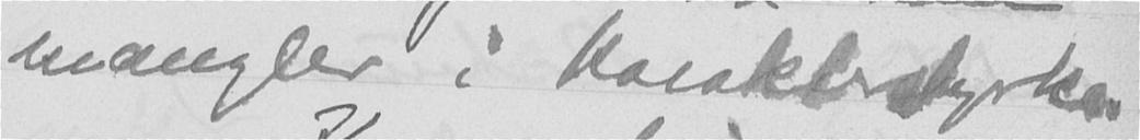mangler i karakterstyrke.
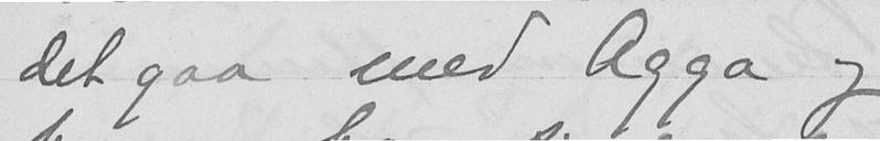det gaa med Agga og
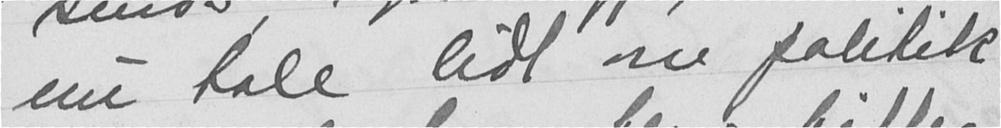nu tale lidt om politik
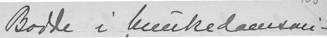Bodde i Munkedamsvei -
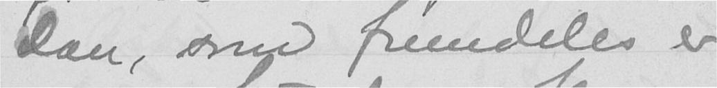Don, som fremdeles er
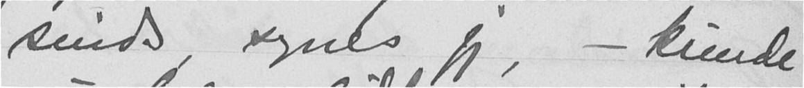sinds, synes jeg, - kunde
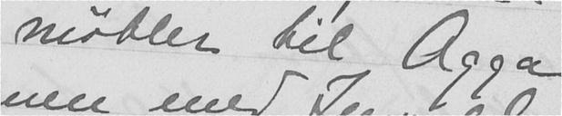møbler til Agga
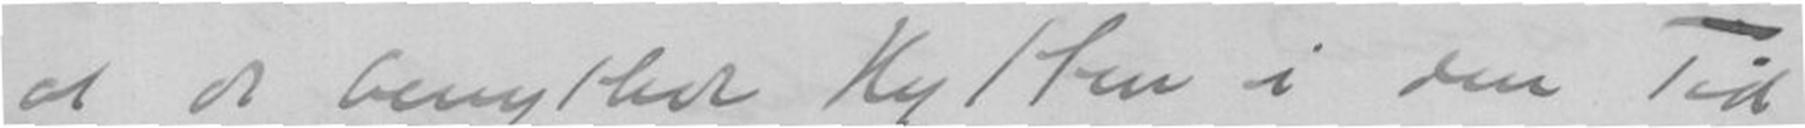at de benyttedt Hytten i den Tid
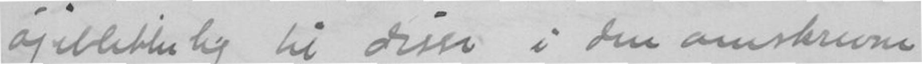øieblikkelig til disse i den omskrevne
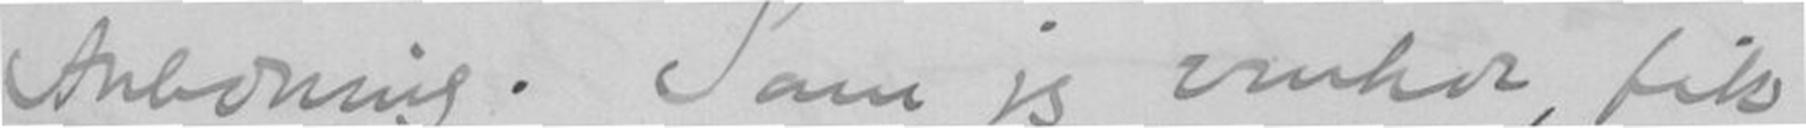Anledning. Som jeg ventet, fik
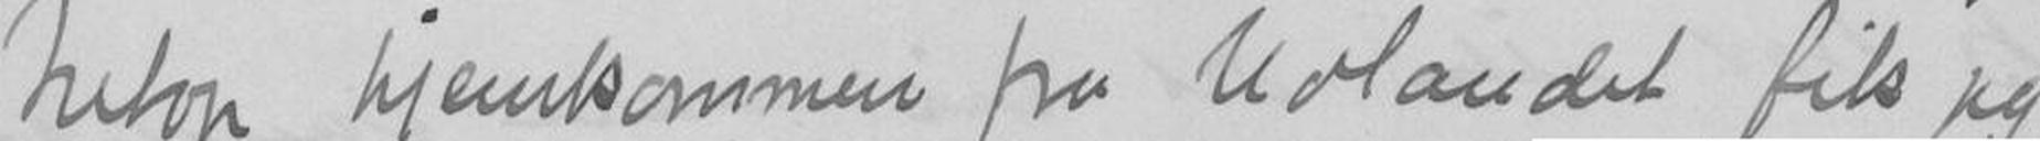Netop hjemkommen fra Udlandet fik jeg
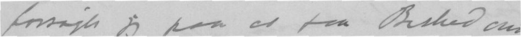forsøgte jeg paa at faa Besked om
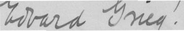Edvard Grieg!
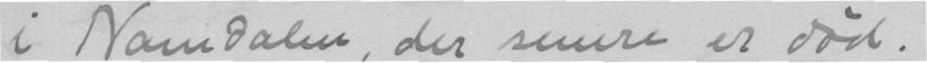i Namdalen, der senere er død.
